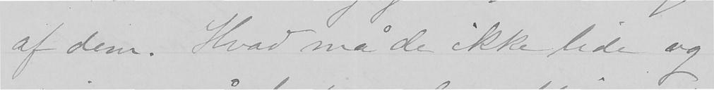af dem. Hvad må de ikke lide og
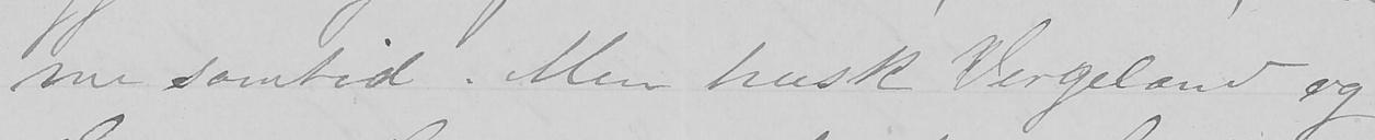me samtid. Men husk Vergeland og
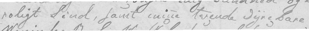roligt Sind, samt mine tvende Dyrebare
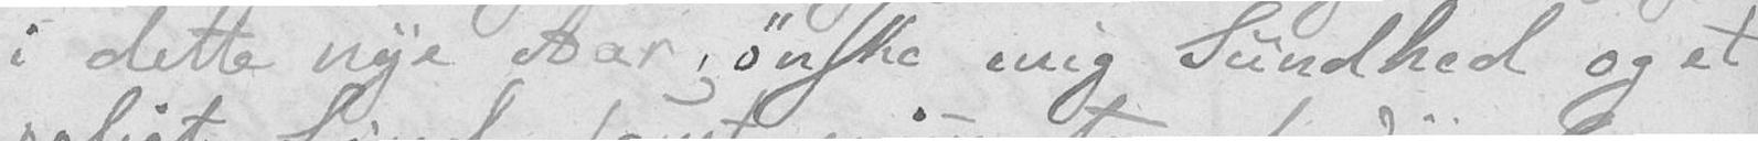i dette nye Aar, ønske mig Sundhed og et
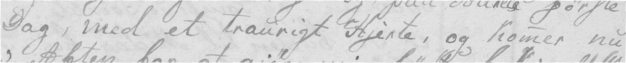Dag, med et traurigt Hjerte, og kommer nu
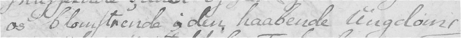os blomstrende i den haabende Ungdomr
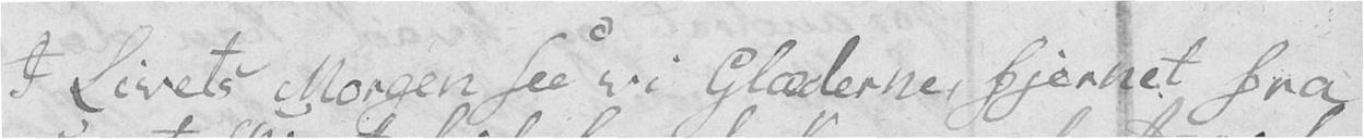I Livets Morgen see vi Glæderne, fjernet fra
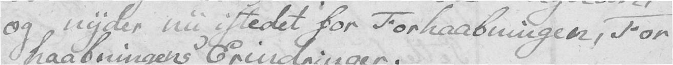og nyder nu istedet for Forhaabningen, For
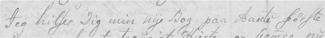Jeg hilser Dig min nye Bog, paa Aarets første
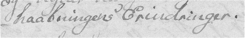-haabningens Erindringer.
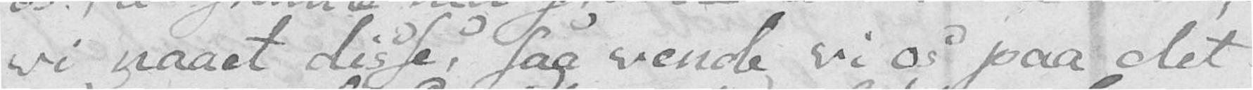vi naaet disse, saa vende vi os paa det
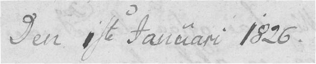Den 1ste Januari 1826.
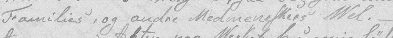Families, og andre Medmeneskers Vel. –
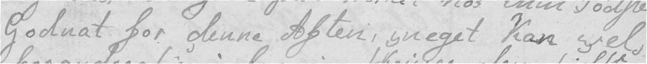Godnat for denne Aften, meget kan vel
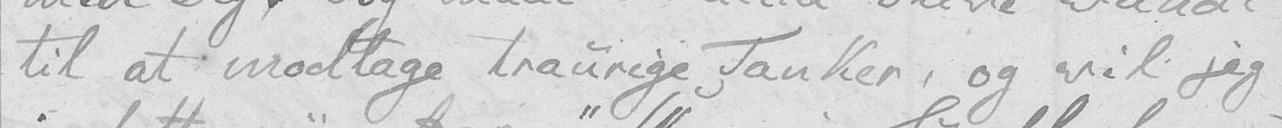til at modtage traurige Tanker, og vil jeg
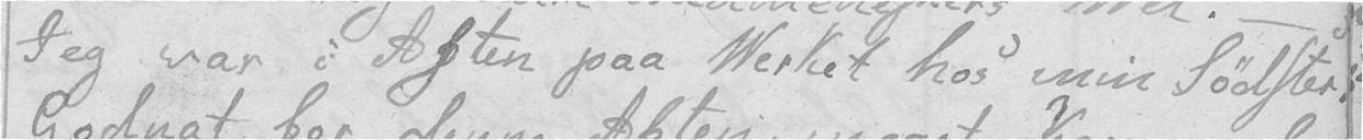Jeg var i Aften paa Verket hos min Sødster.
